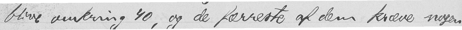blive omkring 40, og de færreste af dem kræve nogen
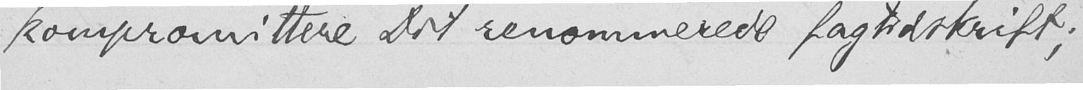kompromittere Dit renommerede fagtidskrift;
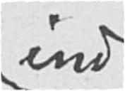ind
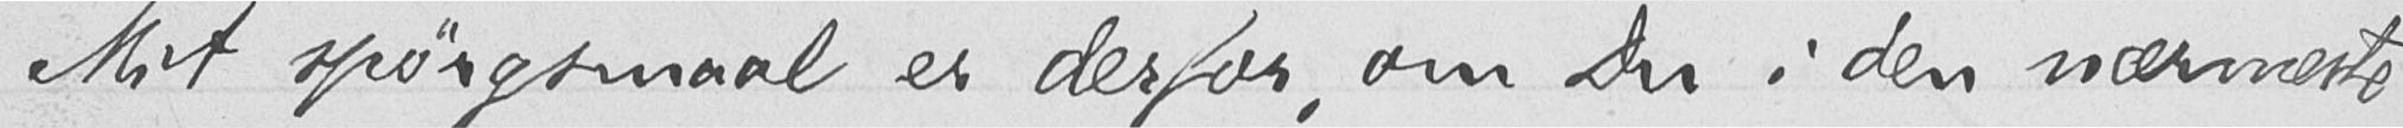Mit spørgsmaal er derfor, om Du i den nærmeste
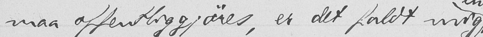maa offentliggjøres, er det faldt mig
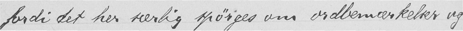fordi det her særlig spørges om ordbemærkelser og
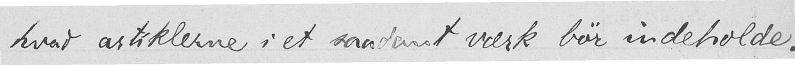hvad artiklerne i et saadant værk bør indeholde.
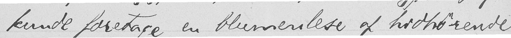kunde foretage en blumenlese af hidhørende
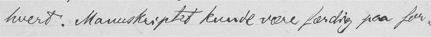hvert. Manuskriptet kunde være færdig paa for-
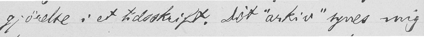gjørelse i et tidsskrift. Dit "arkiv" synes mig
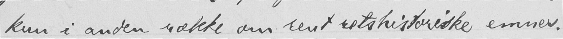kun i anden række om rent retshistoriske emner.
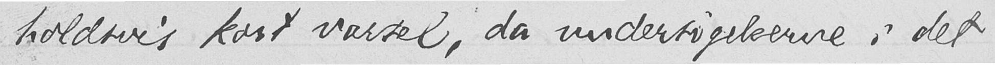holdsvis kort varsel, da undersøgelserne i det
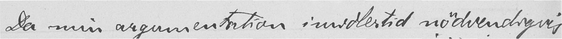Da min argumentation imidlertid nødvendigvis
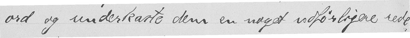ord og underkaste dem en noget udførligere rede-
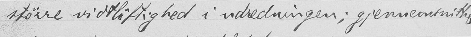større vidtløftighed i udredningen; gjennemsnitlig
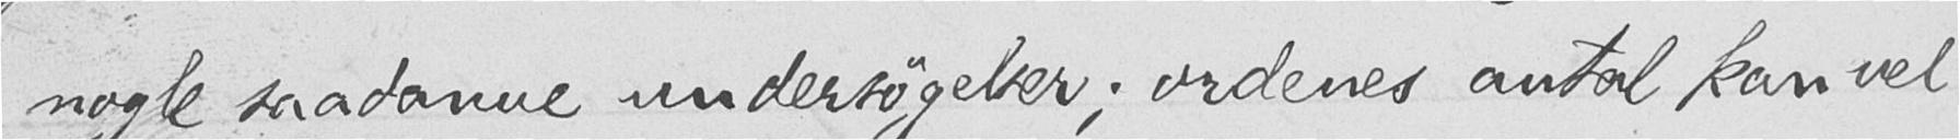nogle saadanne undersøgelser; ordenes antal kan vel
